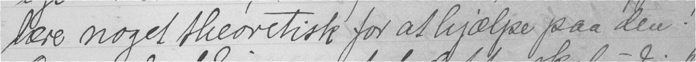lære noget theoretisk for at hjælpe paa den!
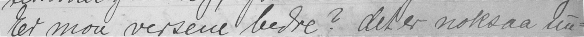Er mon versene bedre? det er noksaa un-
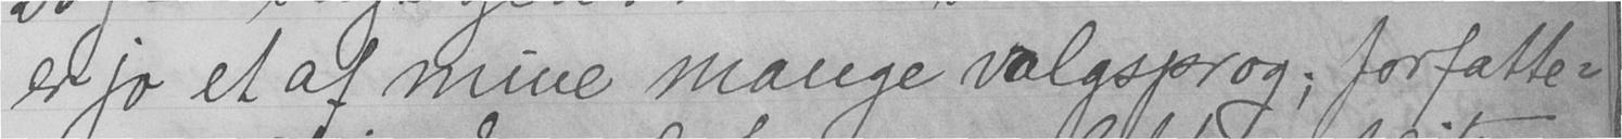er jo et af mine mange valgsprog; forfatte-
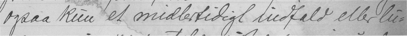ogsaa kun et midlertidigt indfald eller lu-
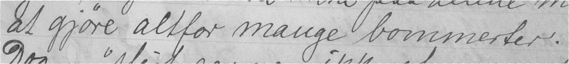at gjøre altfor mange bommerter.
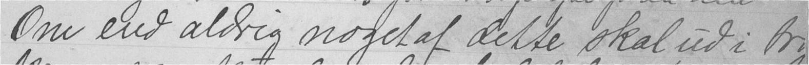Om end aldrig noget af dette skal ud i try
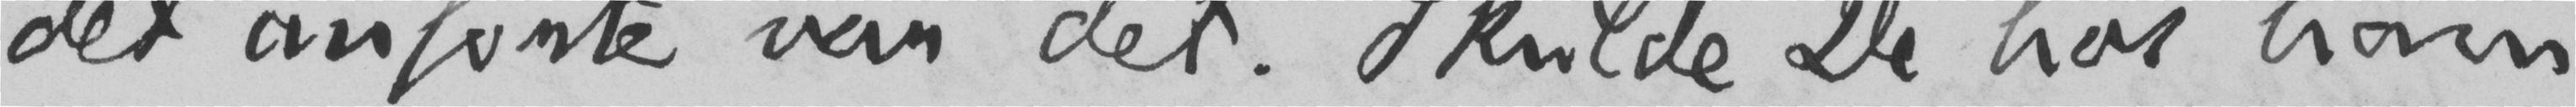det anførte var det. Skulde De hos ham
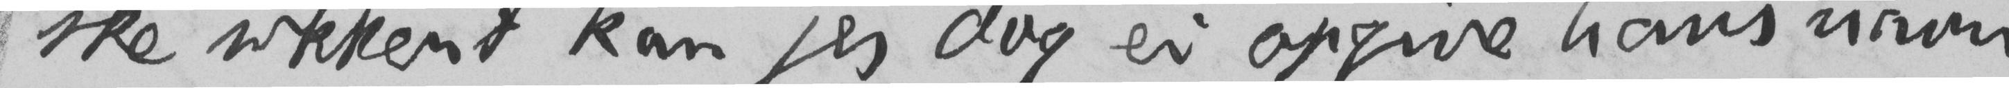ske sikkert kan jeg dog ei opgive hans navn
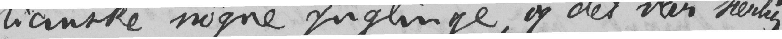lianske nøgne ynglinge, og det var særlig
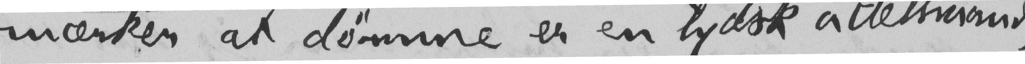mærker at dømme er en tydsk adelsman,
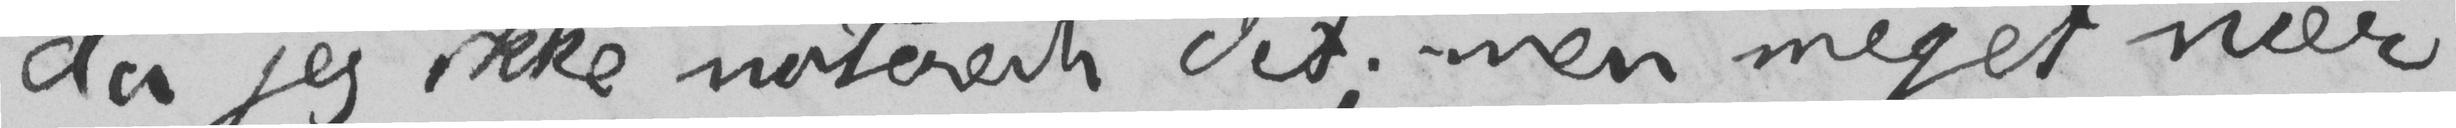da jeg ikke noterede det; men meget nær
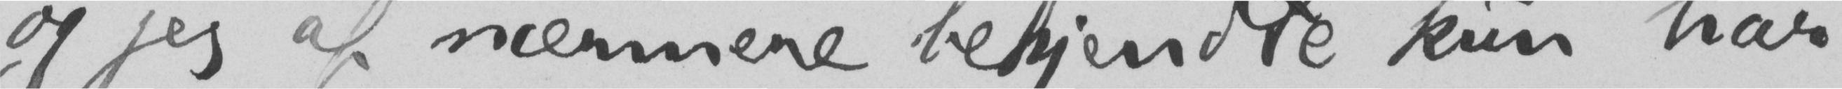og jeg af nærmere bekjendte kun har
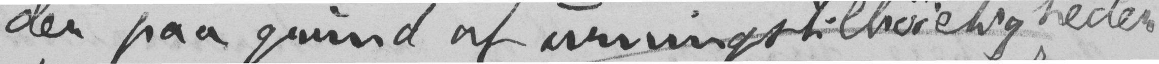der paa grund af urningstilbøieligheder
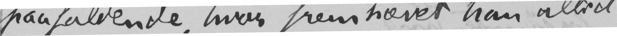paafaldende, hvor fremhævet han altid
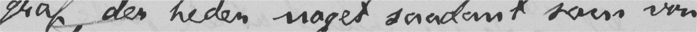graf, der heder noget saadant som von
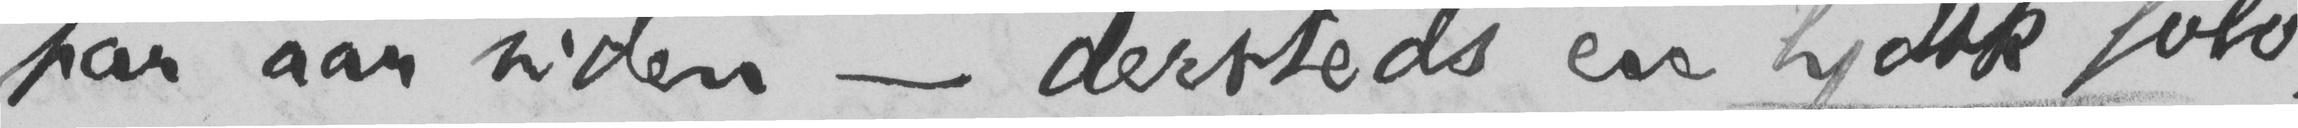par aar siden – dersteds en tydsk foto-
